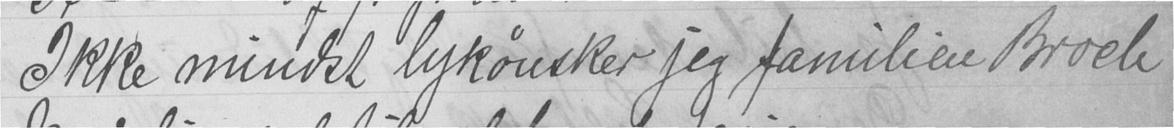Ikke mindst lykønsker jeg familien Broch
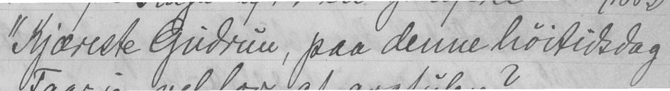"Kjæreste Gudrun, paa denne høitidsdag
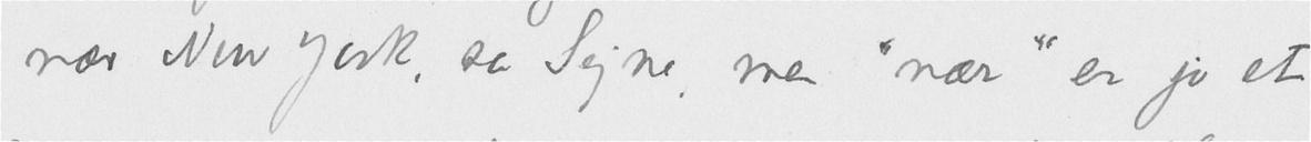nær New York, sa Signe, men "nær" er jo et
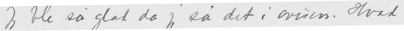Jeg ble så glad da jeg så det i avisen.  Hvad
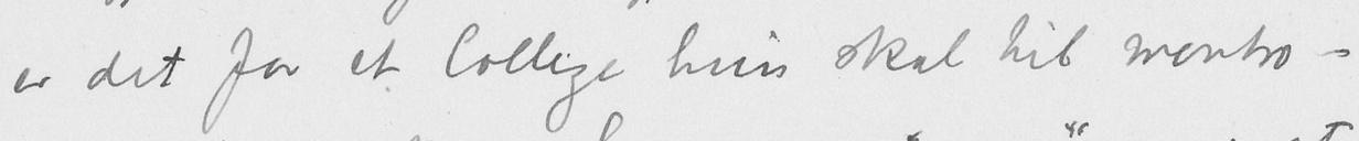er det for et College hun skal til montro –
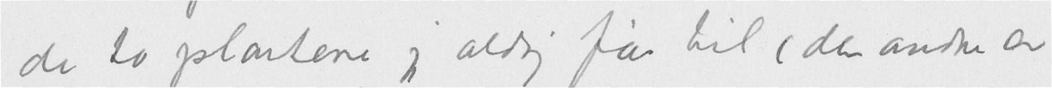de to plantene jeg aldrig får til (den andre er
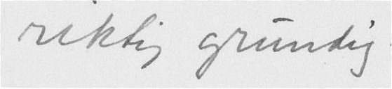riktig grundig.
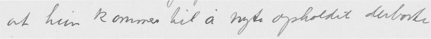at hun kommer til å nyte opholdet derborte
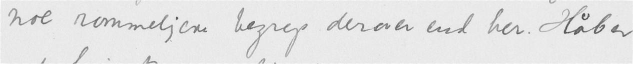noe rommeligere begrep derover end her.  Håber
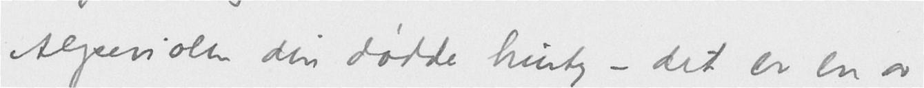Alpeviolen din dødde hurtig – det er en av
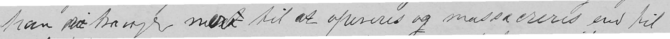han vi trænger mere til at opereres og massacreres end til
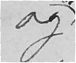og
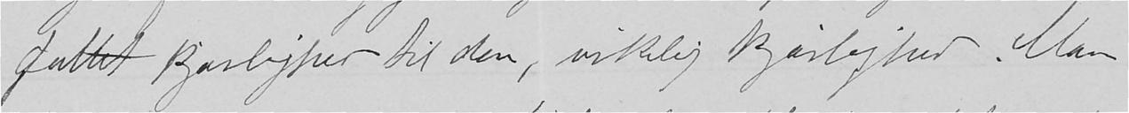fattet kjærlighed til den, virkelig kjærlighed. Man
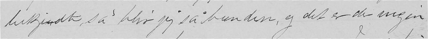bekjendte, så blir jeg så bunden, og det er der ingen
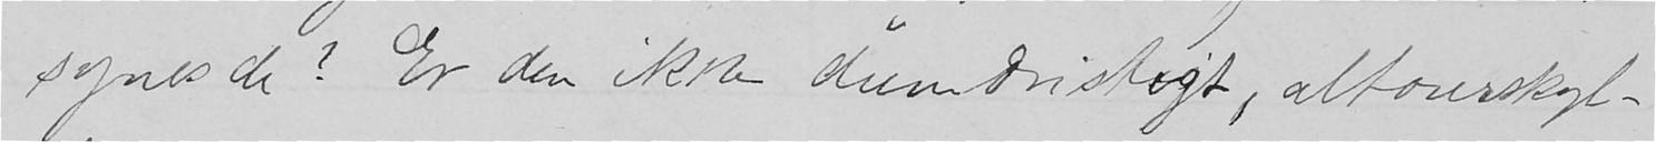synes de? Er den ikke dumdristigt, altoverskyl-
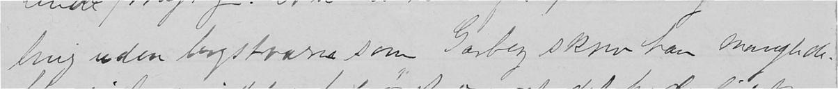ling uden brystværn som Garborg skrev han manglede.
